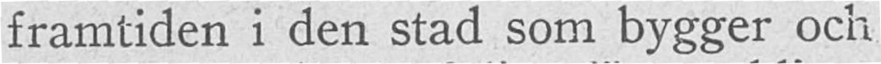framtiden i den stad som bygger och
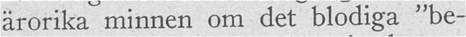ärorika minnen om det blodiga "be-
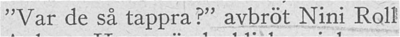"Var de så tappra?" avbröt Nini Roll
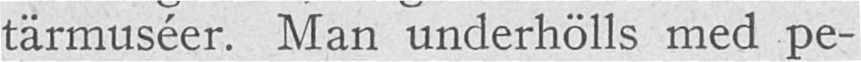tärmuséer. Man underhölls med pe-
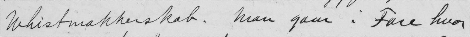Whistmakkerskab. Man gaar i Fare hvor
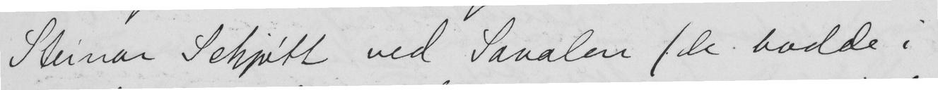Steinar Schjøtt ved Savalen (de bodde i
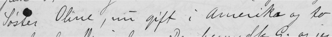Søster Oline, nu gift i Amerika og to
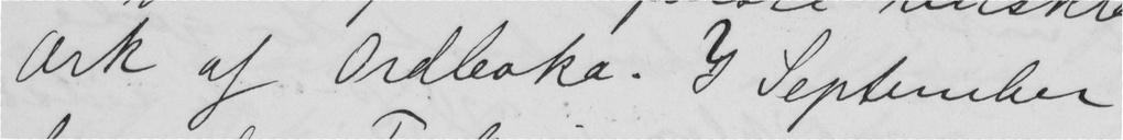Ark af Ordboka. I September
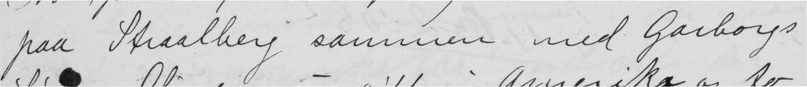paa Straalberg sammen med Garborgs
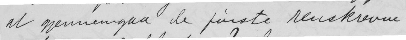at gjennemgaa de første renskrevne
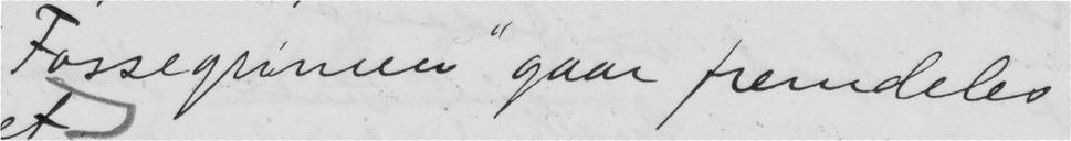"Fossegrimen" gaar fremdeles
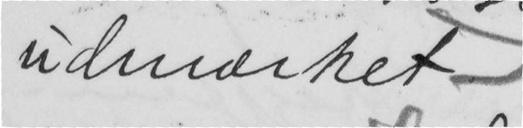udmærket—
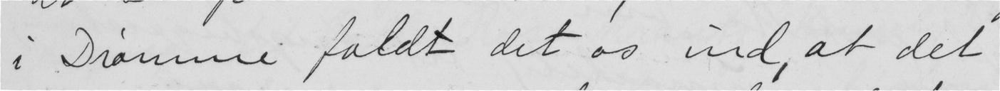i Drømme faldt det os ind, at det
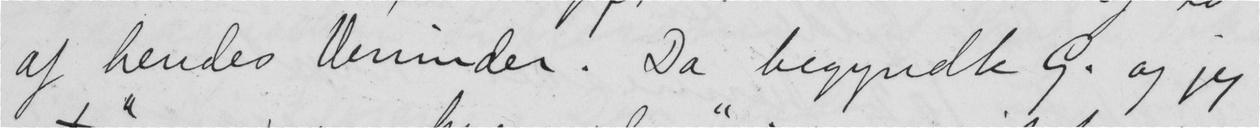af hendes Veninder. Da begyndte G. og jeg
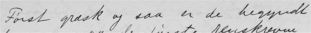Først græsk og saa er de begyndt
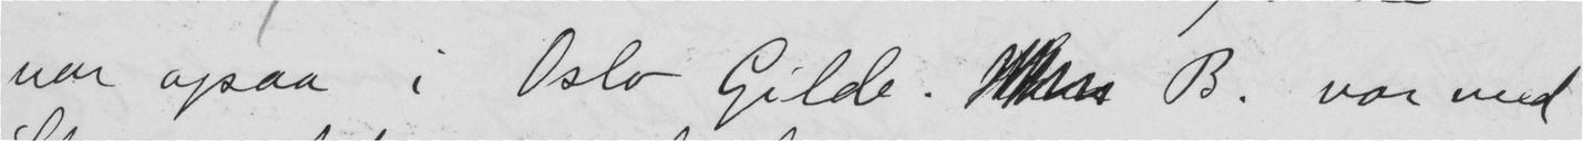var ogsaa i Oslo Gilde.  B. var med
